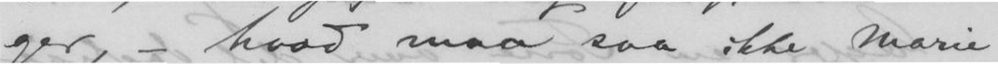ger, - hvad maa saa ikke Marie
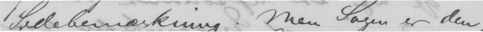Sidebemærkning. Men Sagen er den,
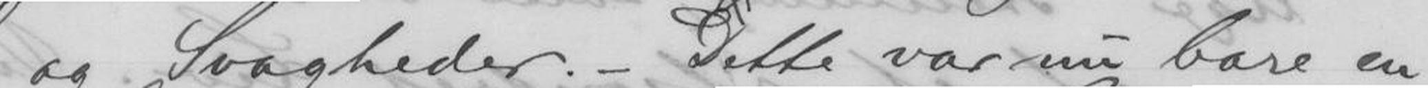og Svagheder. - Dette var nu bare en
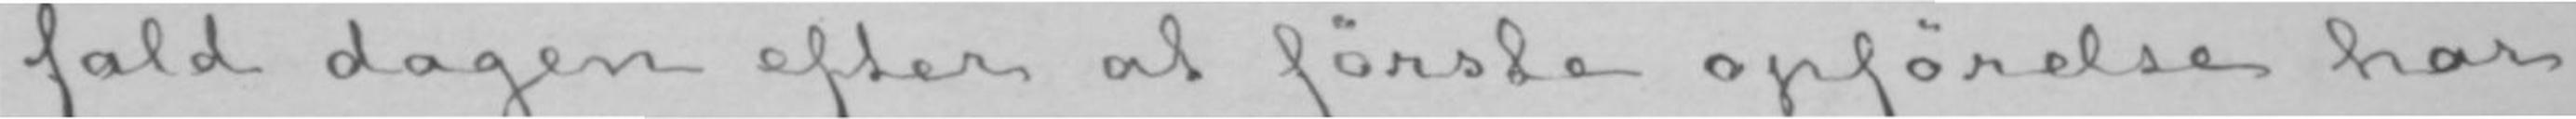fald dagen efter at første opførelse har
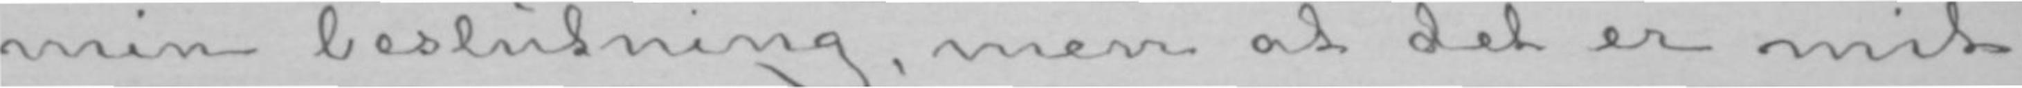min beslutning, men at det er mit
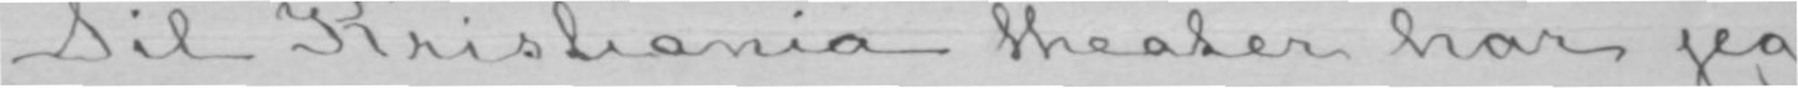Til Kristiania theater har jeg
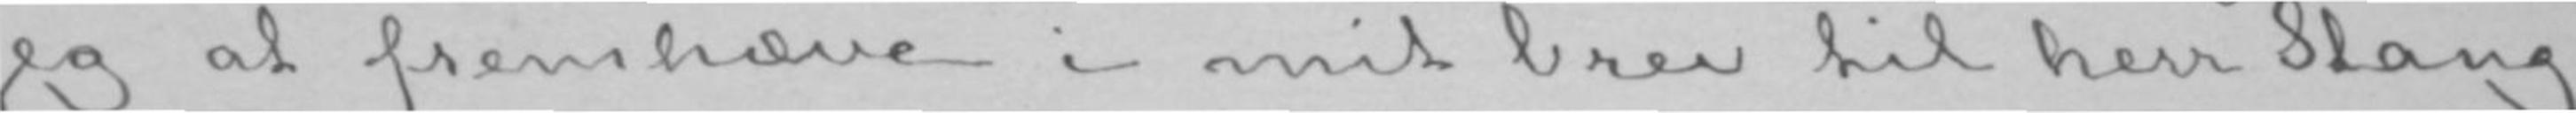jeg at fremhæve i mit brev til herr Stang
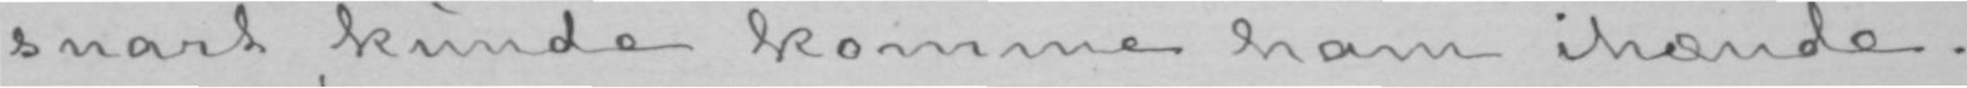snart kunde komme ham ihænde.
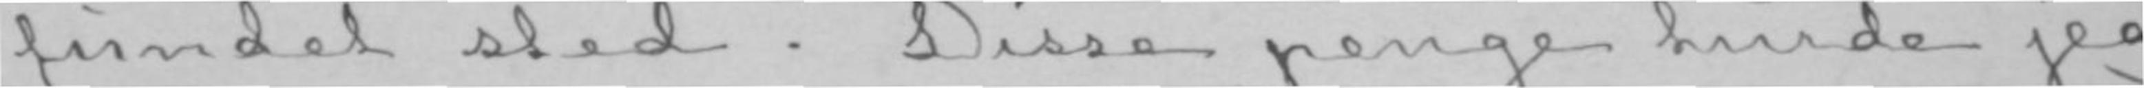fundet sted. Disse penge turde jeg
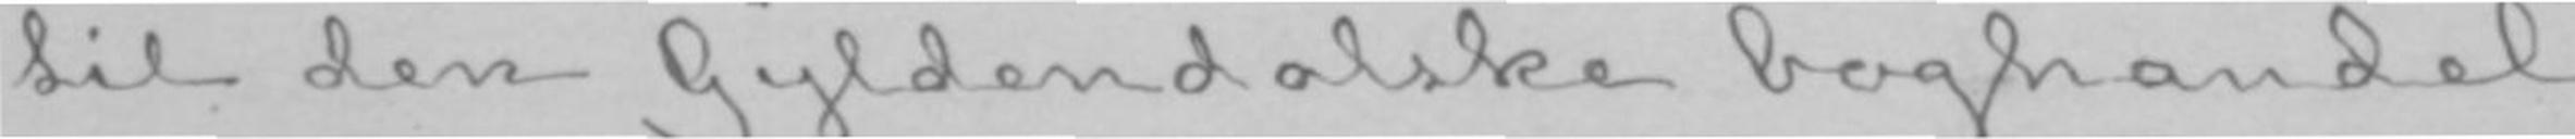til den Gyldendalske boghandel.
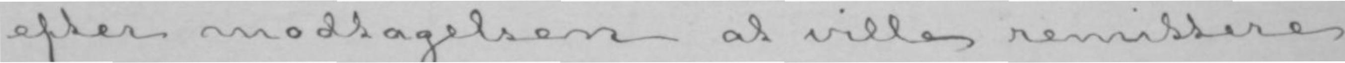efter modtagelsen at ville remittere
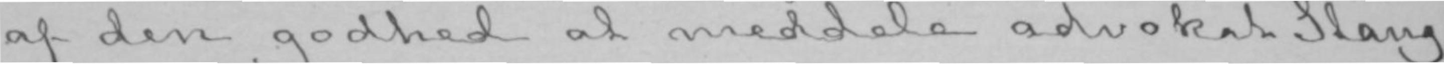af den godhed at meddele advokat Stang
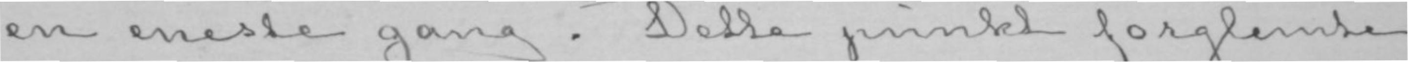en eneste gang. Dette punkt forglemte
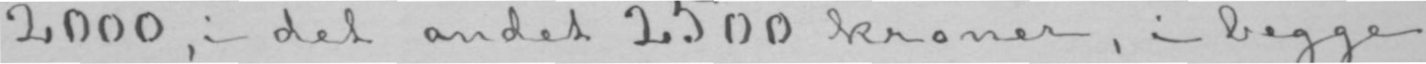2000, i det andet 2500 kroner, i begge
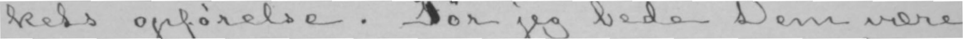kets opførelse. Tør jeg bede Dem være
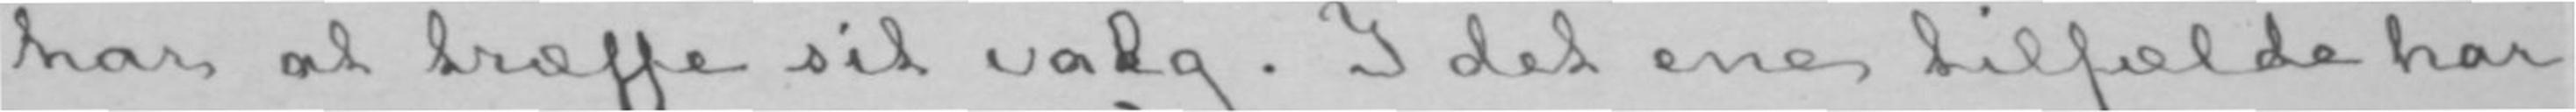har at træffe sit valg. I det ene tilfælde har
